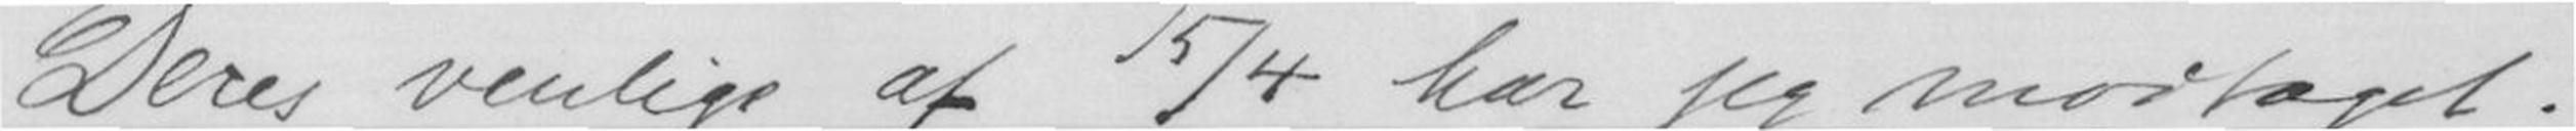Deres venlige af 15/4 har jeg modtaget.
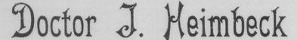Doctor J. Heimbeck
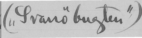("Svanø bugten")
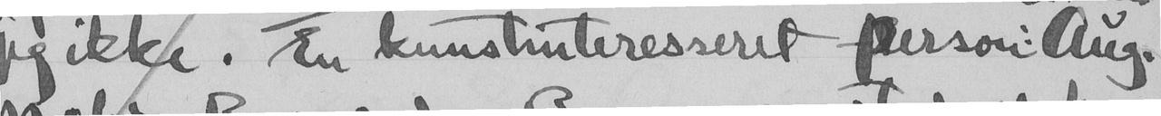jeg ikke. En kunstinteresseret person: Aug.
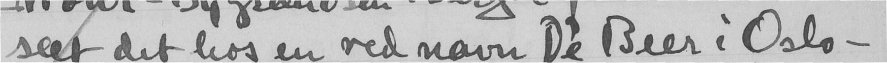seet det hos en ved navn De Beer i Oslo -
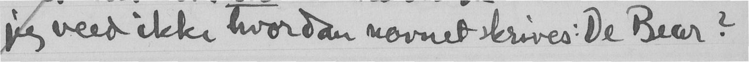jeg veed ikke hvordan nøvnet skrives: De Bear?
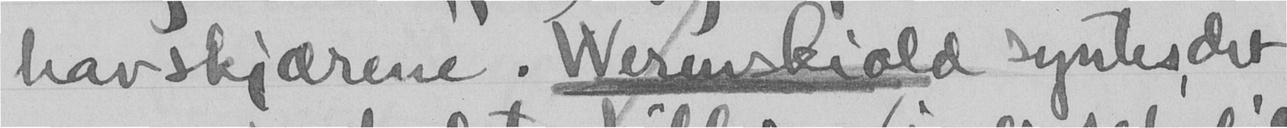havskjærene. Werenskiold syntes, det
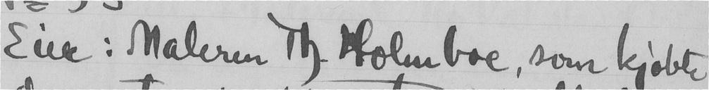Eier: Maleren Th. Holmboe, som kjøbte
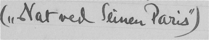("Nat ved Seinen Paris")
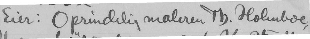Eier: Oprindelig maleren Th. Holmboe,
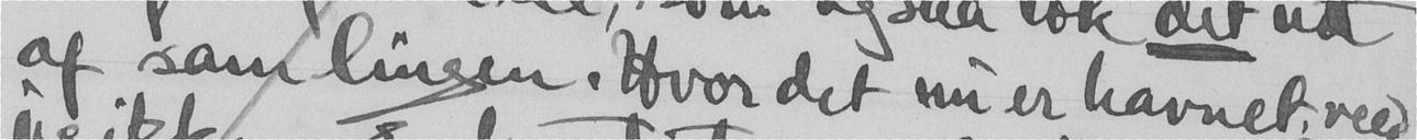af samlingen. Hvor det nu er havnet veed
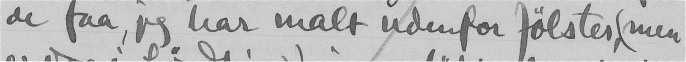de faa, jeg har malt udenfor Jølster, (men
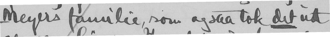Meyers familie, som ogsaa tok det ut
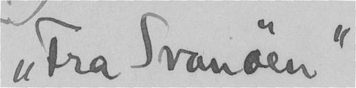"Fra svanøen"
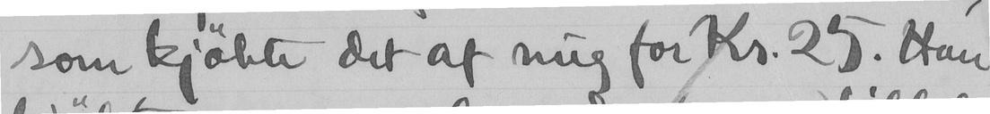som kjøbte det af mig for Kr. 25. Han
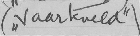("Vaarkveld!")
